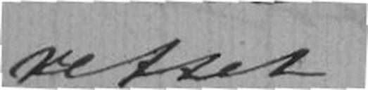rettet
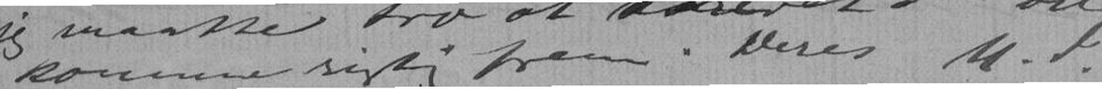komme rigtig frem. Deres H. I.
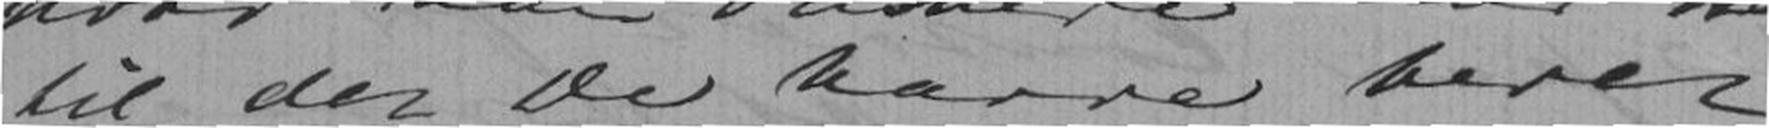til det de havde bedet
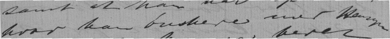hvad han buskede med Hensyn
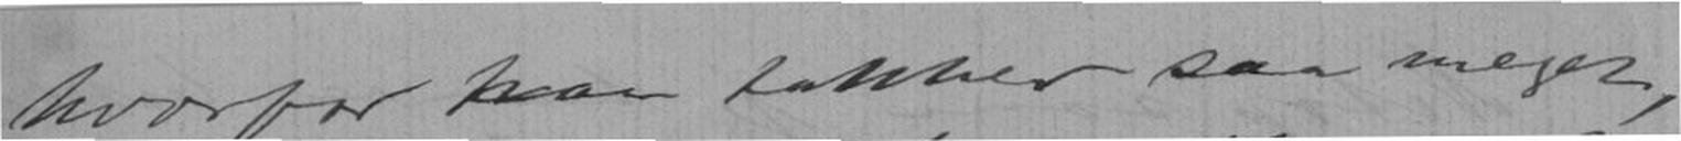hvorfor han takker saa meget,
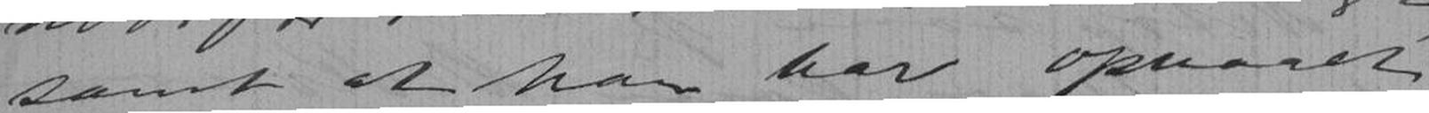samt at han har opnaaet
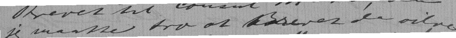jeg maaske vil at Brevet da vilde
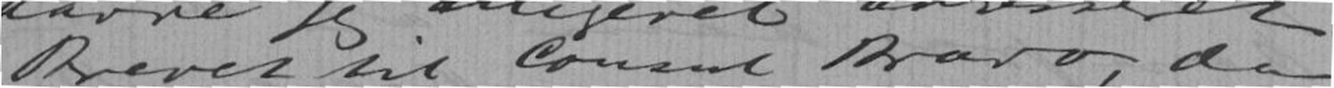Brevet til Consul Bravo, da
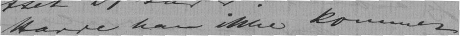Havde han ikke kommet
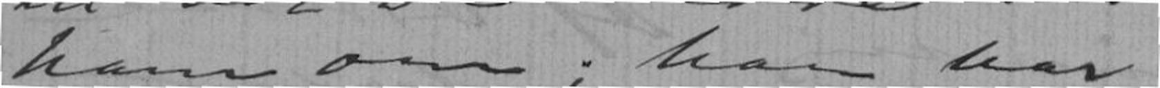ham om; han har
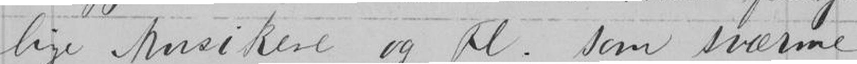lige Musikere og Fl. som sværme
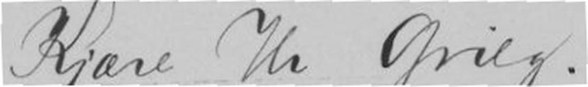Kjære Hr Grieg.
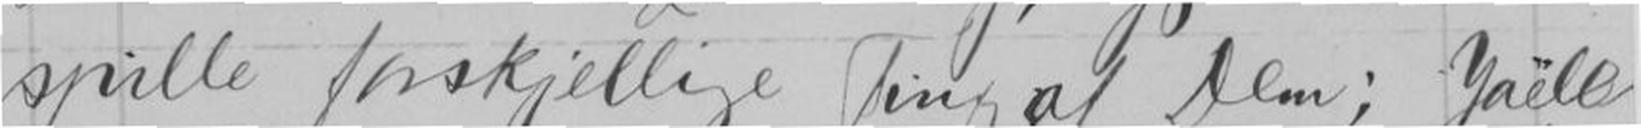spille forskjellige Ting af Dem; Jaéle
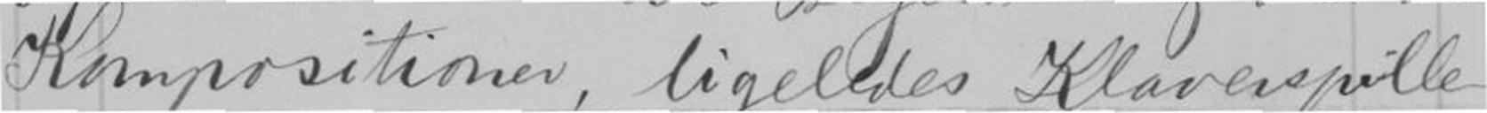Kompositioner, ligeledes Klaverspille-
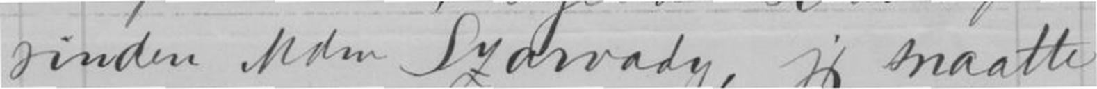rinden Mdm Szawady, jeg maatte
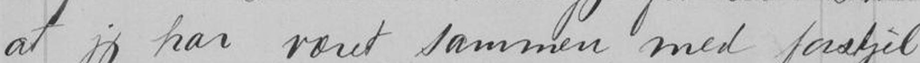at jeg har været sammen med forskjel-
